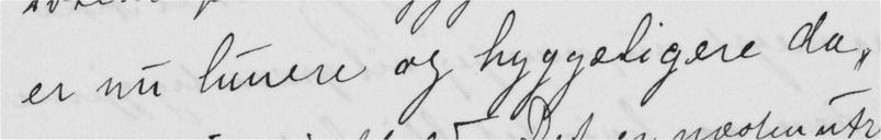er nu lunere og hyggeligere da,
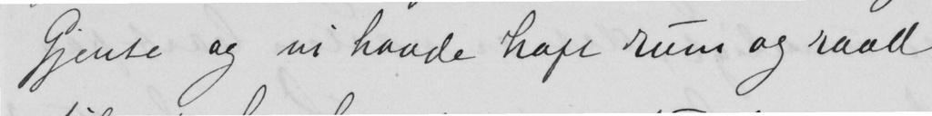Tjente og vi havde hafe sum og raal
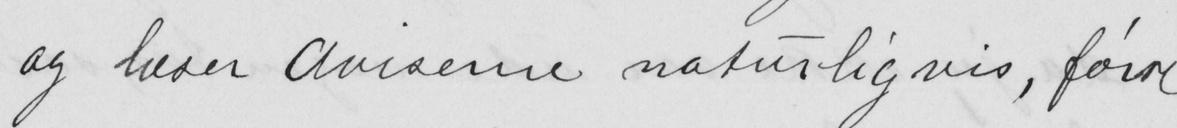og læser aviserne naturligvis, førre
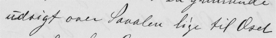udsigt over Savalen lige til Æsel
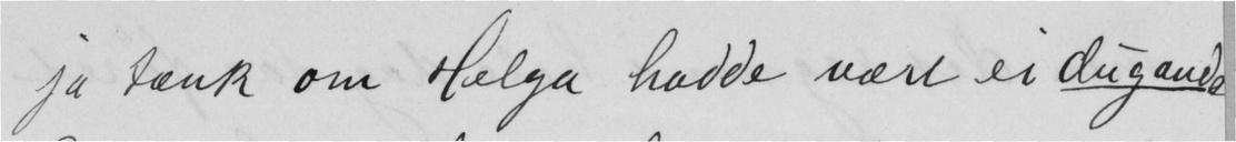ja tænk om Helga hadde vært ei dugaande
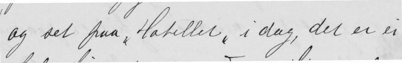og det paa. Hotellet i dag, det er ei
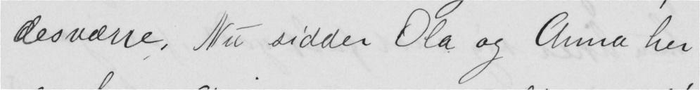desværre. Nu sidder Ola og Anna her
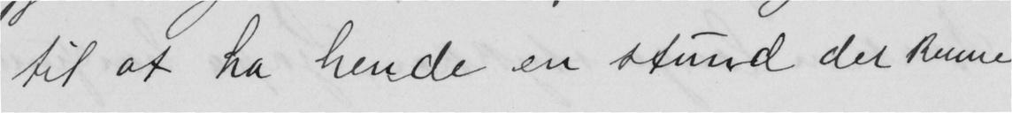til at ha hende en stund det Rune
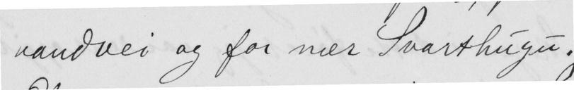vandvei og for nær Svarthùgù.
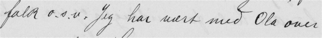folk o.s.v. Jeg har vært med Ola over
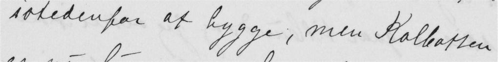istedenfor at bygge, men Kolbotten
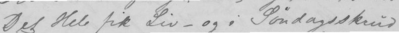Det Hele fik Liv - og i Søndagsskrud
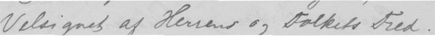Velsignet af Herrens og Folkets Fred;
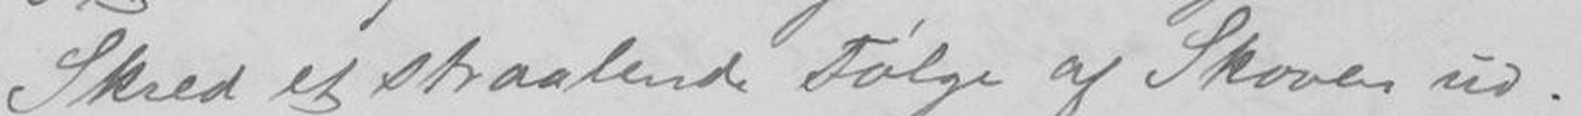Skred et straalende Følge af Skoven ud!:
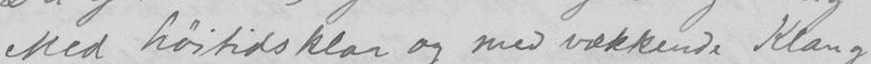Med høitidsklar og med vækkende Klang,
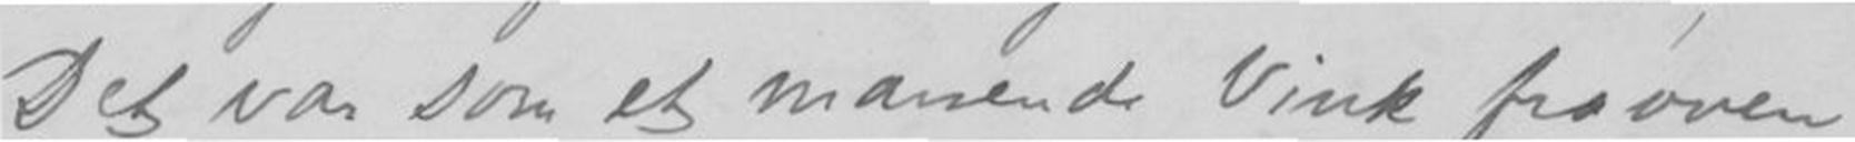Det var som et manende Vink fra oven
